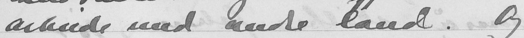arbeide med andre land. Og
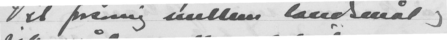Vil forening mellom landsmål og
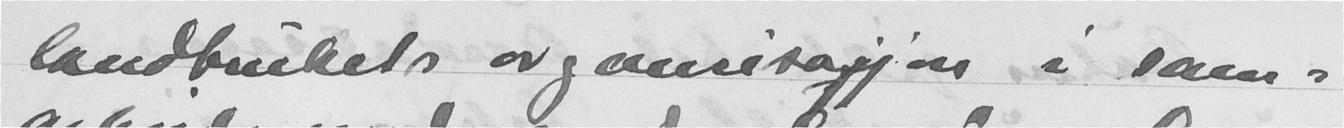landbrukets organisasjon i sam-
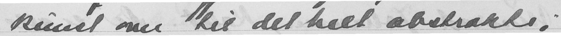kunst over til det helt abstrakte;
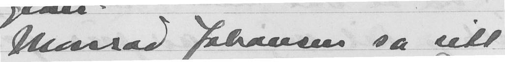Monrad Johansen, sa sitt
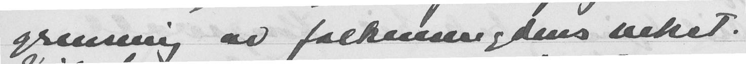grensnig av folkemengdens enket.
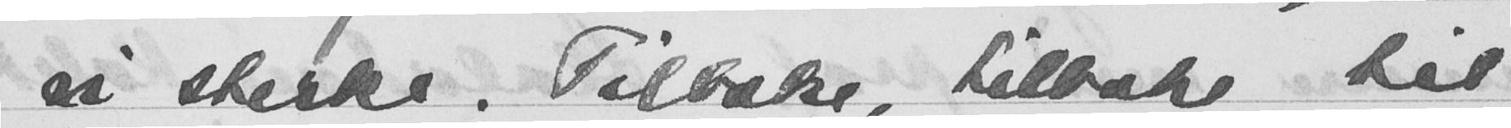vi sterke. Tilbake, tilbake til
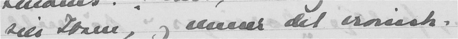sier Ibsen, og mener det ironisk.
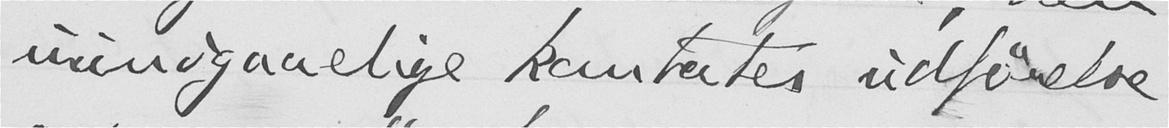uundgaaelige kantates udførelse
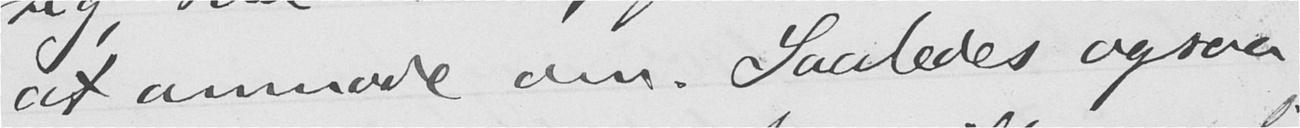at anmode om. Saaledes ogsaa
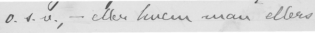o. s. v., - eller hvem man ellers
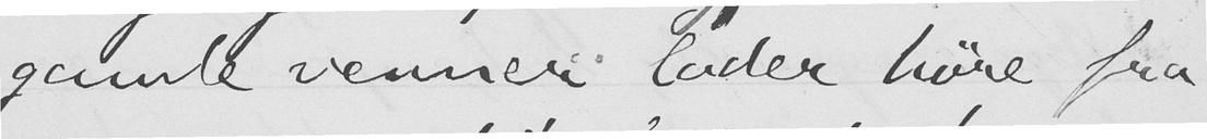gamle venner lader høre fra
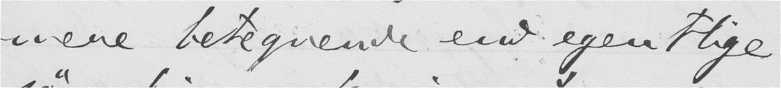mere betegnende end egentlige
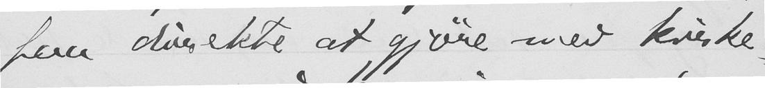faa direkte at gjøre med kirke-
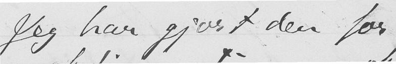Jeg har gjort den for
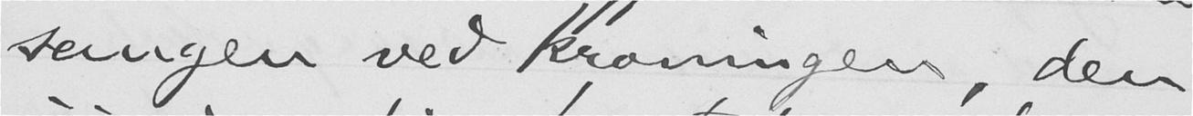sangen ved kroningen, den
